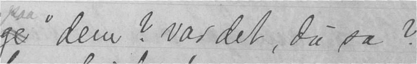ge" dem? var det, du sa?
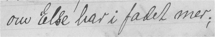om Else har i fadet mer;
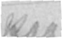paa
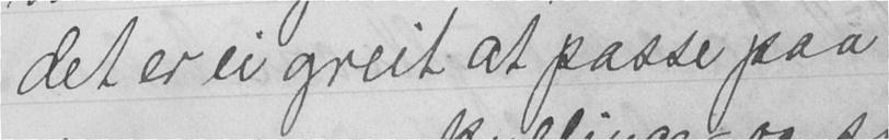det er ei greit at passe paa
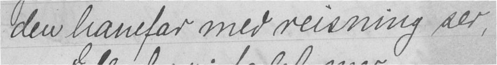den hanefar med reisning ser,
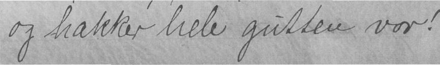og hakker hele gutten vor!
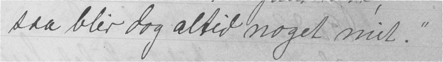saa blir dog altid noget mit."
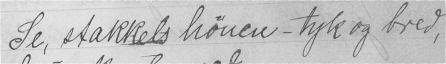Se, stakkels hønen - tyk og bred,
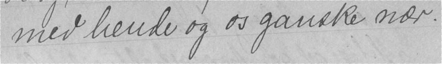med hende og os ganske nær.
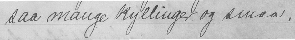saa mange kyllinger og smaa.
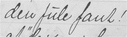den fule fant!
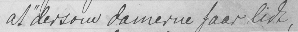at dersom damerne faar lidt,
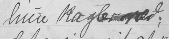hun kagler ræd;
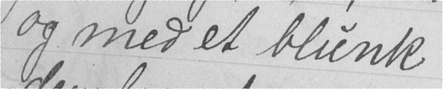og med et blunk
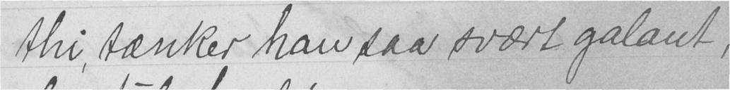thi, tænker han saa svært galant,
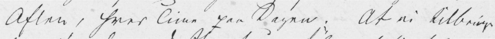Aften, hver Time paa Dagen. At vi tilbringe
